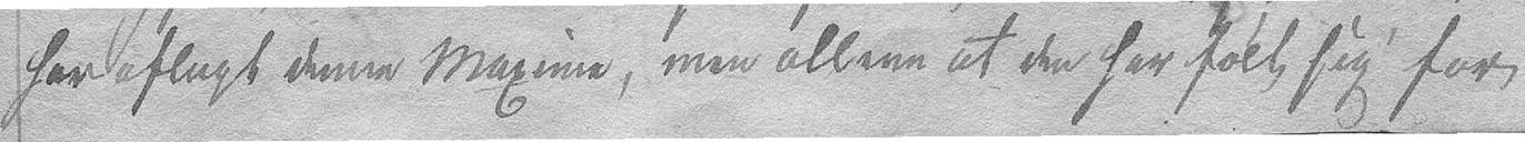har aflagt denne Maxime, men allene at den har følt sig for
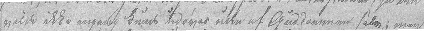vilde ikke engang kunde udøves uden af Guddommen selv; men
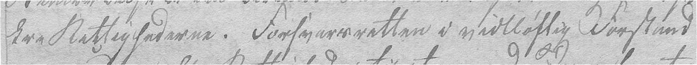kre Rettighederne. Forsvarsretten i vidtløftig Forstand
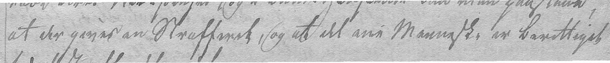at der gives en Strafferet, og at det ene Menneske er berettiget
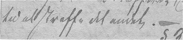til at straffe det andet. –
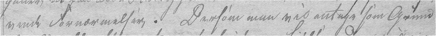vende Fornærmelser. Dersom man vil antage som Grund
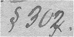§ 302.
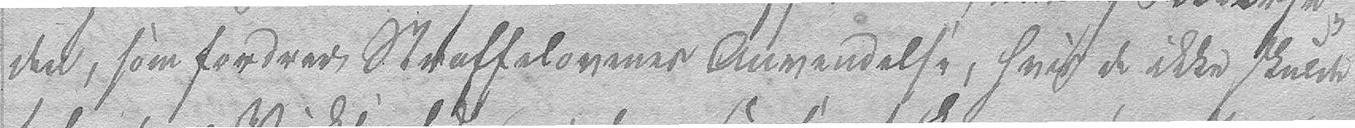den, som fordrer Straffelovenes Anvendelse, hvis de ikke skulde
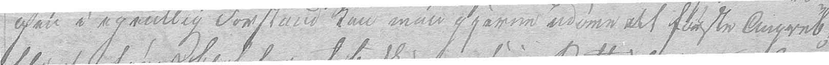gen i egentlig Forstand kan man gjerne udøve det første Angreb;
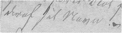deraf sit Navn. –
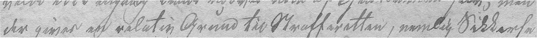der gives en relativ Grund til Strafferetten, nemlig Sikkerhe-
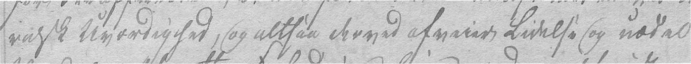ralsk Uværdighed, og altsaa derved afveier Lidelse og uædel
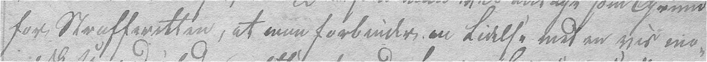for Strafferetten, at man forbinder en Lidelse med en vis mo-
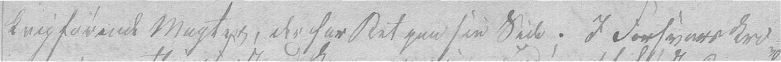krigførende Magter, der har Ret paa sin Side. I Forsvarskri-
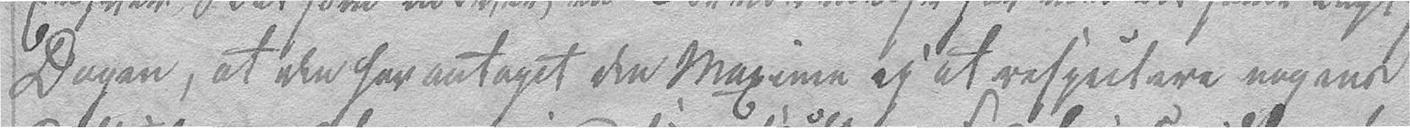Dagen, at den har antaget den Maxime ey at respectere nogen
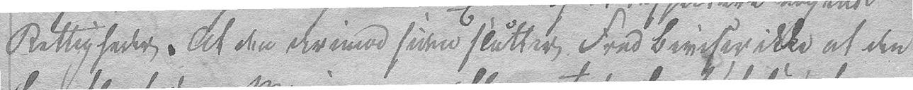Rettigheder. At den derimod siden slutter Fred beviser ikke at den
